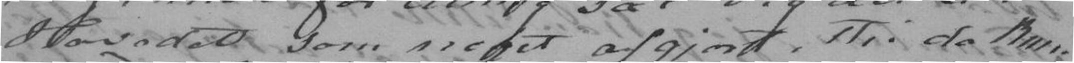Hovedet som noget afgjort, thi da kun-
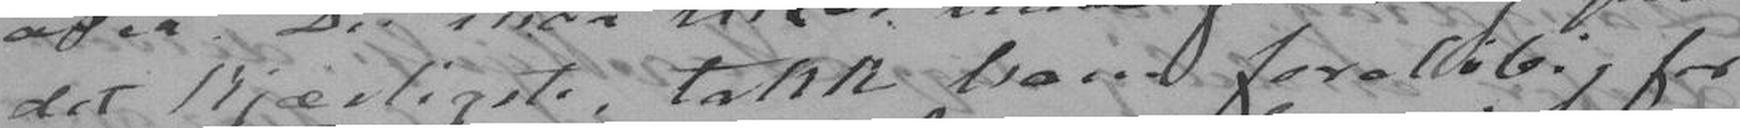det kjærligste, takke ham foreløbig for
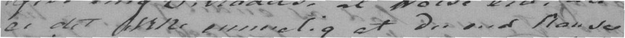er det ikke umuelig at Du end kan se
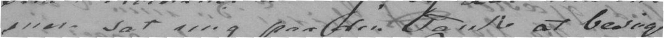mere sat mig paa den Tanke at besøge
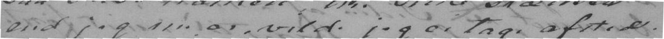end jeg nu er vilde jeg ei tage afsted.
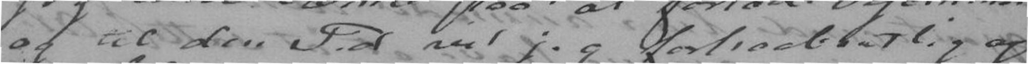og til den Tid vil jeg forhaabentlig og-
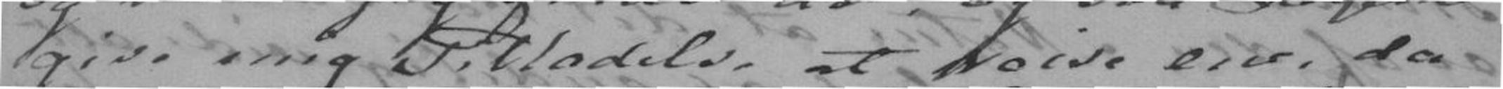give mig Tilladelse at reise ene, da
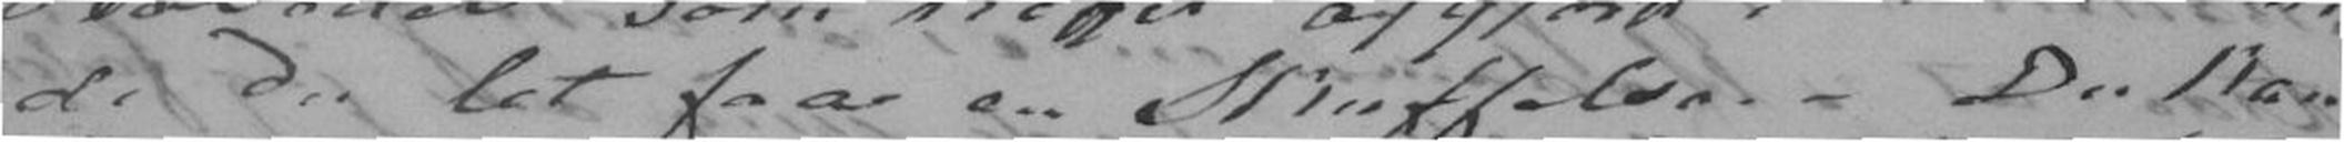de Du let faae en Skuffelse. - Du kan
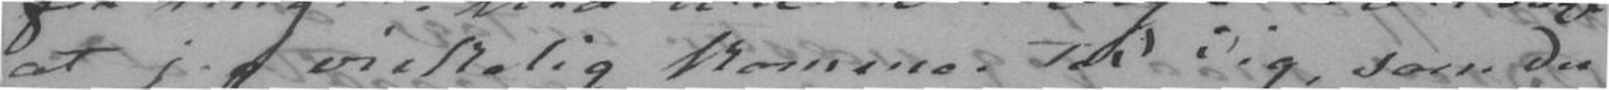at jeg virkelig kommer til Dig, som Du
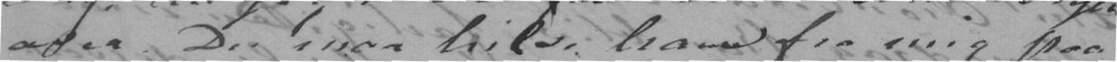over. Du maa hilse ham fra mig paa
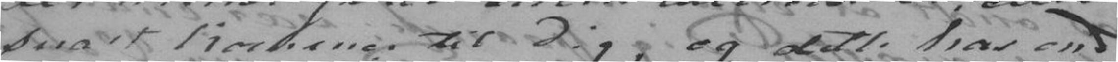snart kommer til Dig, og dette har end
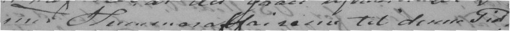med Hummeraffairen til denne Tid,
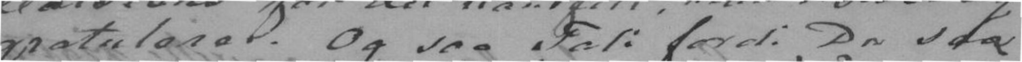gratulerer. Og saa Tak fordi Du saa
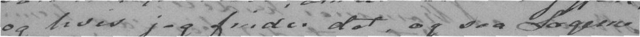og hvis jeg finder det, og saa Lægerne
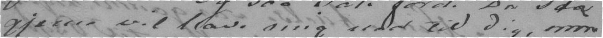gjerne vil have mig ned til Dig, men
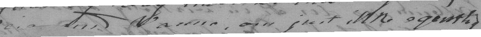med Varme, om just ikke egentlig
